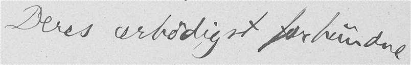Deres ærbødigst forbundne
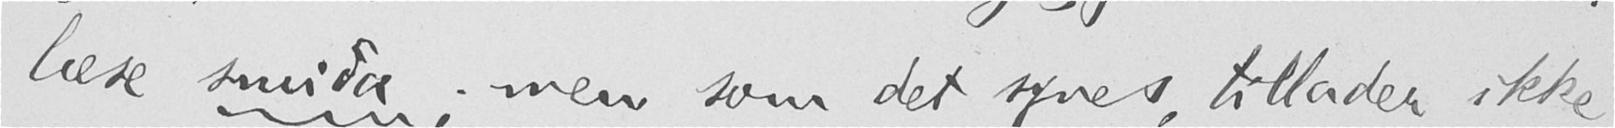læse smiða; men som det synes, tillader ikke
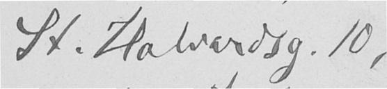St. Halvardsg. 10,
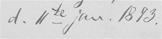d. 11te jan. 1893.
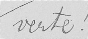verte!
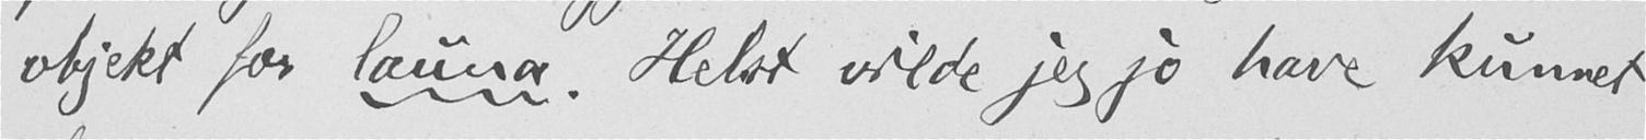objekt for launa. Helst vilde jeg jo have kunnet
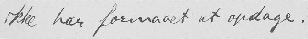ikke har formaaet at opdage.
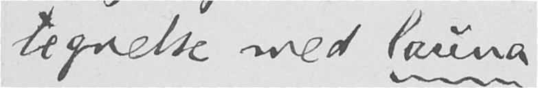tegnelse med launa.
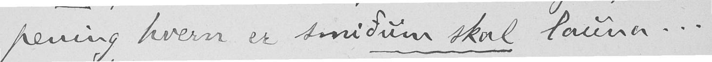pening hvem er smiðum skal launa...
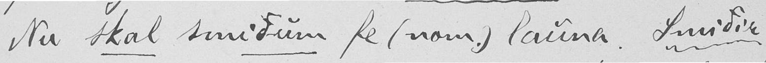Nu skal smiðum fe (nom) launa. Smiðir
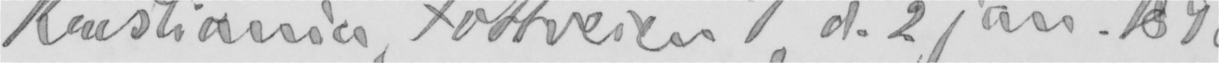Kristiania, Fossveien 7, d. 2. jan. 1898.
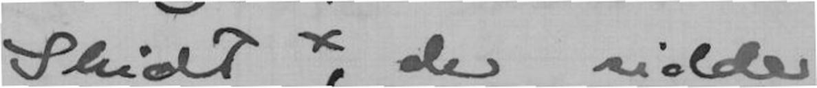Skidt X, der sidder
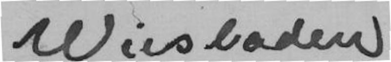Wiesbaden
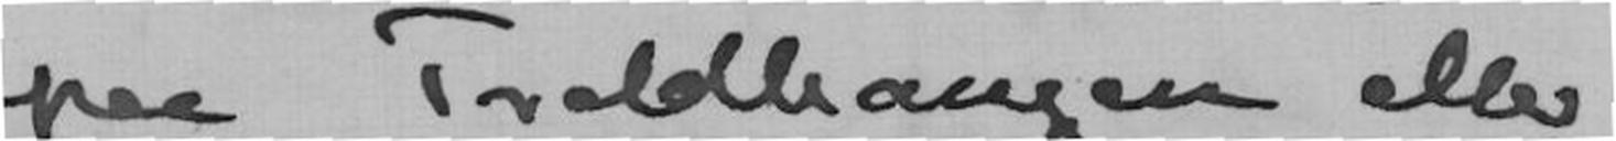paa Troldhaugen eller
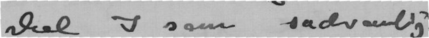skal I som sædvanligt
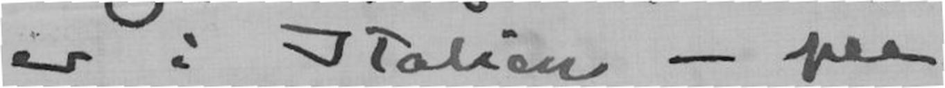er i Italien - paa
